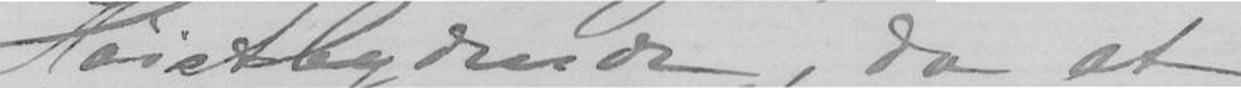Høistbydende, da at
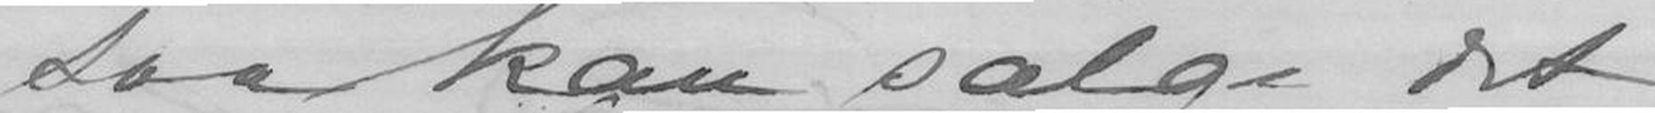saa kan sælge det
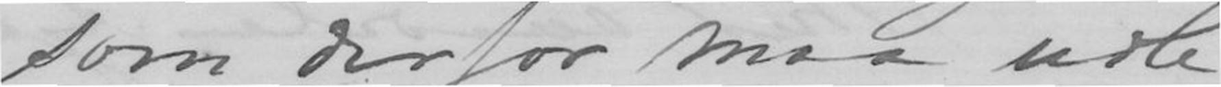som derfor maa udle-
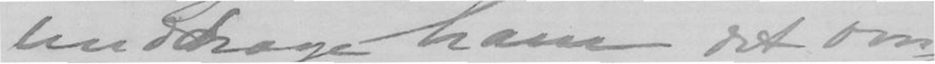unddrage ham det om
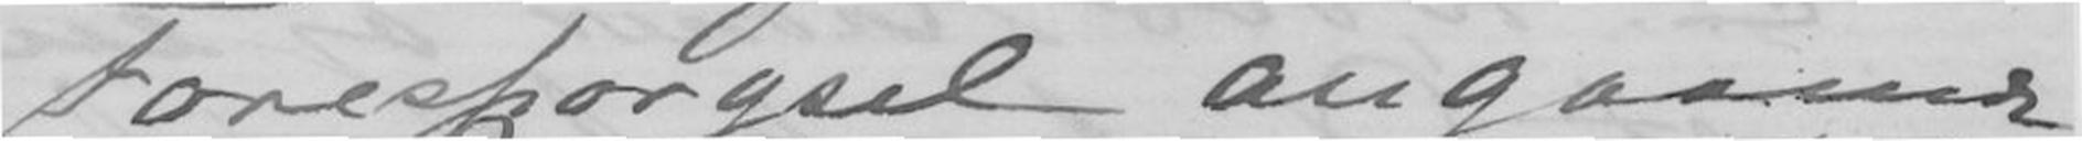Forespørgsel angaaende
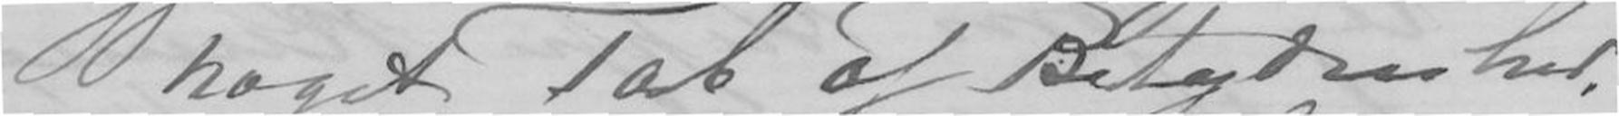noget Tab af Betydenhed,
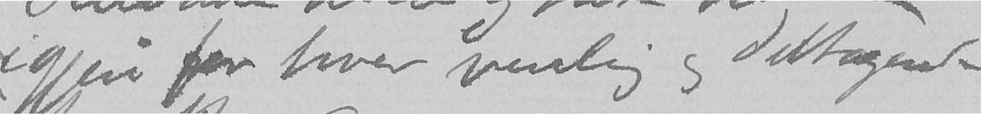igjen for hver venlig og deltagende
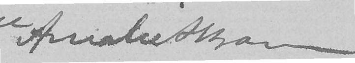Amalie Skram
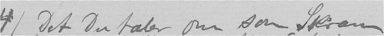4) Det Du taler om som Skram
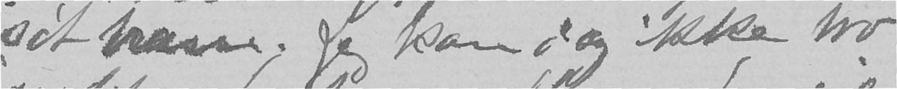sét ham. Jeg kan dog ikke tro
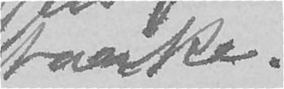tanke.
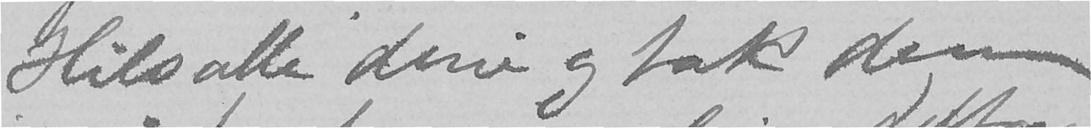Hils alle dine og tak dem
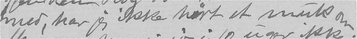med, har jeg ikke hørt et muk om.
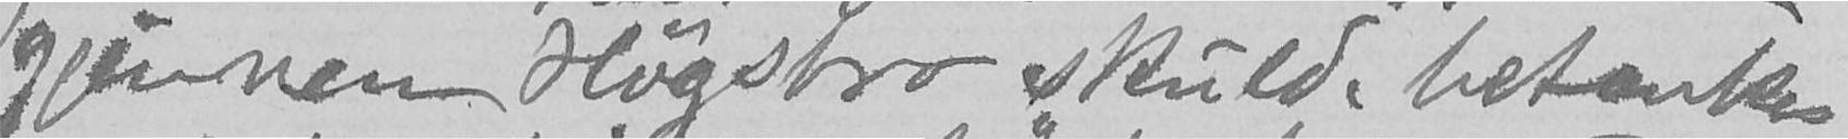gjennem Högsbro skulde betænkes
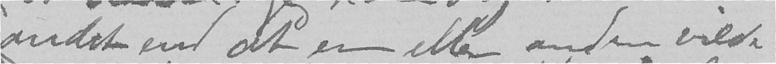andet end at en eller anden vilde
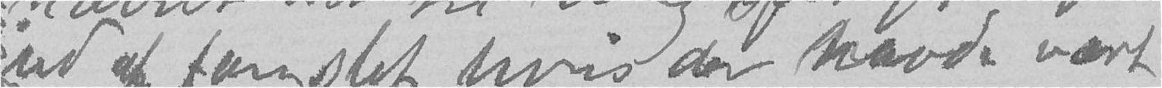ud af fængslet, hvis der havde vært
